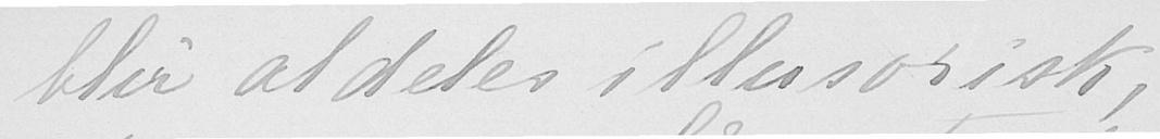blir aldeles illusorisk,
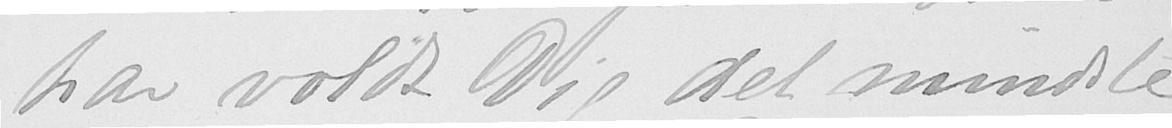har voldt Dig det mindste
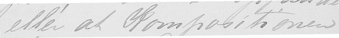eller at Kompositionen
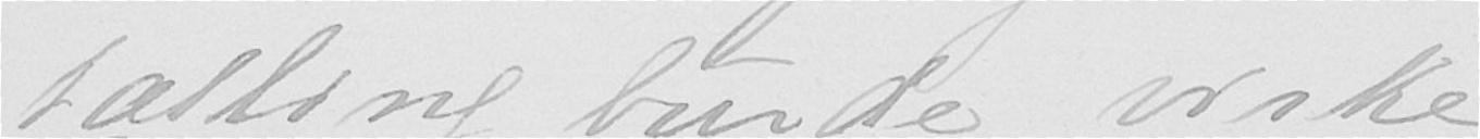tælling burde virke.
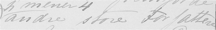andre store Forfattere
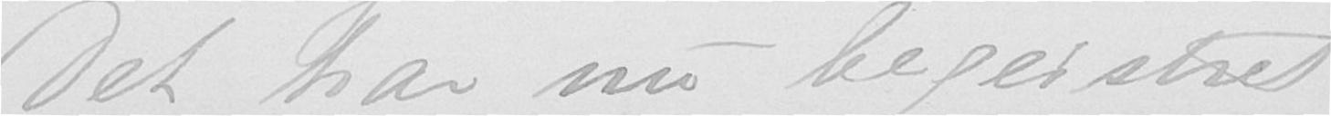Det har nu begeistret
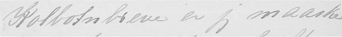Kolbolnbreve er jeg maaske
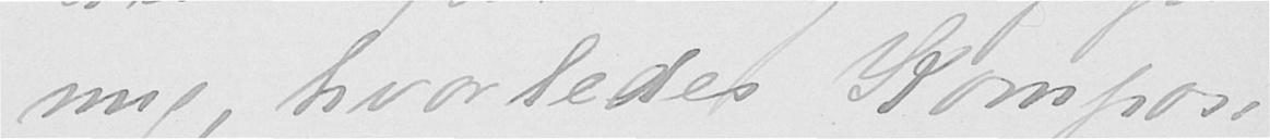mig, hvorledes Komposi-
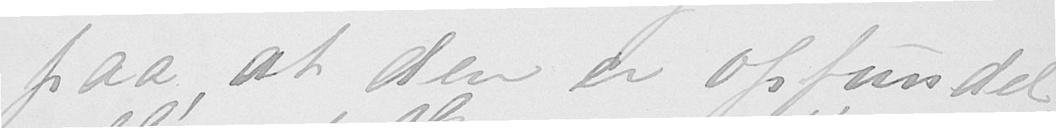paa, at den er opfundet,
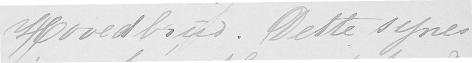Hovedbrud. Dette synes
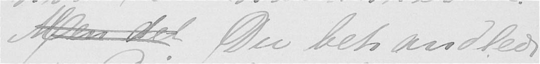Men det Du behandlede
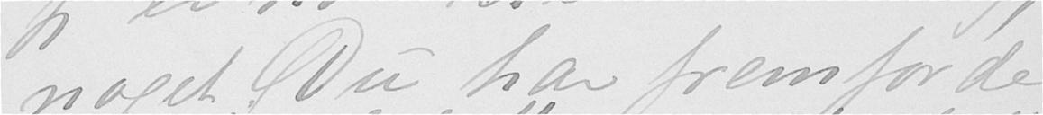noget Du har fremfor de
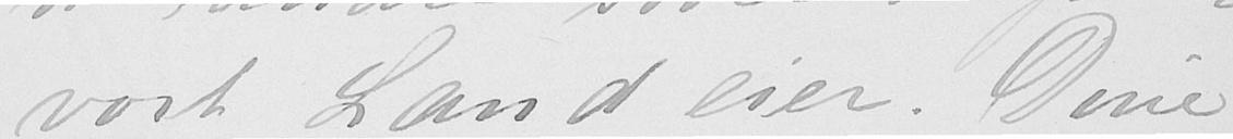vort Land eier. Dine
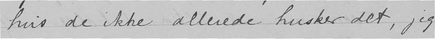hvis de ikke allerede husker det, jeg
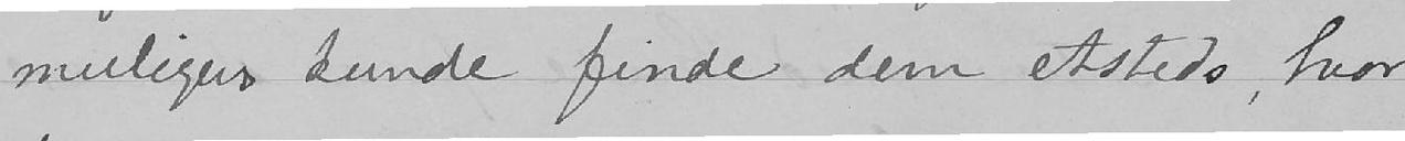muligens kunde finde dem etsteds, hvor
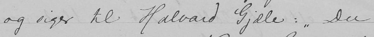og siger til Halvard Gjæle: «Du
